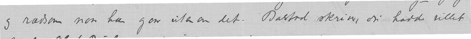og rædsom naar han gaar utenom det. Balstad skriver, du hadde villet
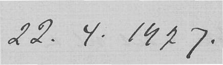22.4.1927.
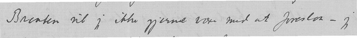Braaten vil jeg ikke gjerne være med at foreslaa - jeg
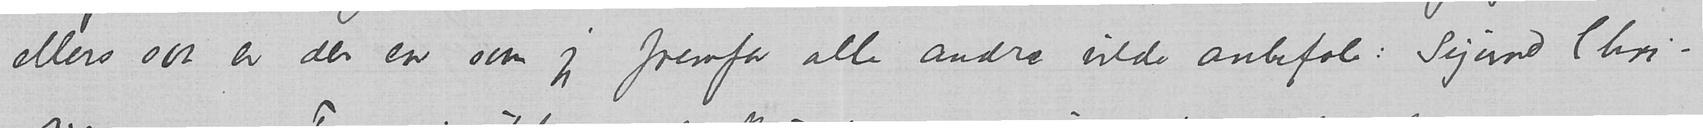ellers saa er der en som jeg fremfor alle andre vilde anbefale: Sigurd Chris -
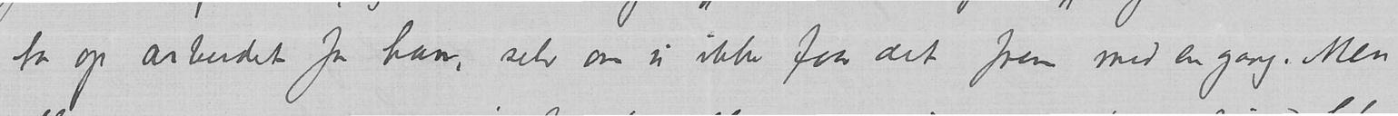ta op arbeidet for han, selv om vi ikke faa det frem med en gang. Men
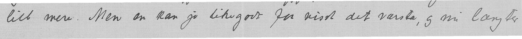litt mere. Men en kan jo likegodt faa visst det værste, og nu længter
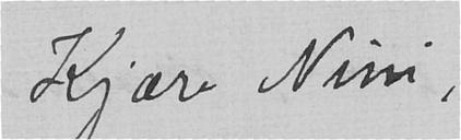Kjære Nini,
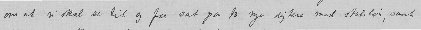om at vi skal se til og faa sat paa to nye digtere med statsløn, samt
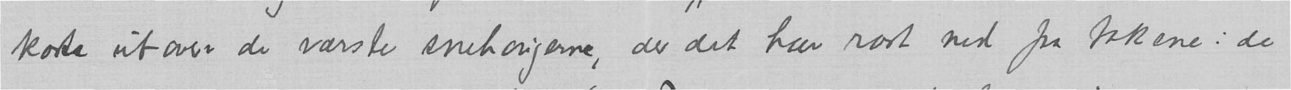koste utover de værste snehaugerne, der det har rast ned fra takene: de
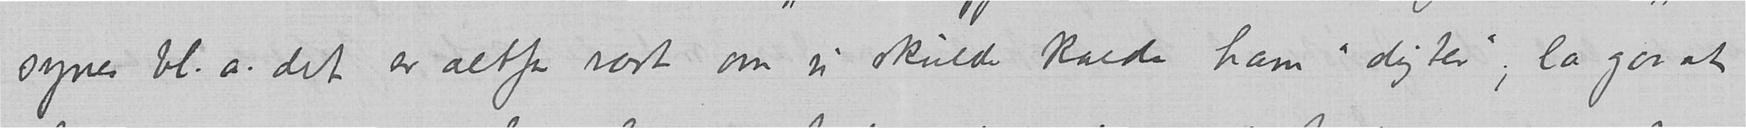synes bl.a. det er altfor rart om vi skulde kalde ham «digter»; la gaa at
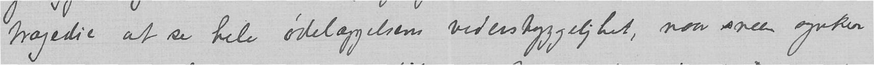tragedie at se hele ødelæggelsens vederstyggelighet, naar sneen synker
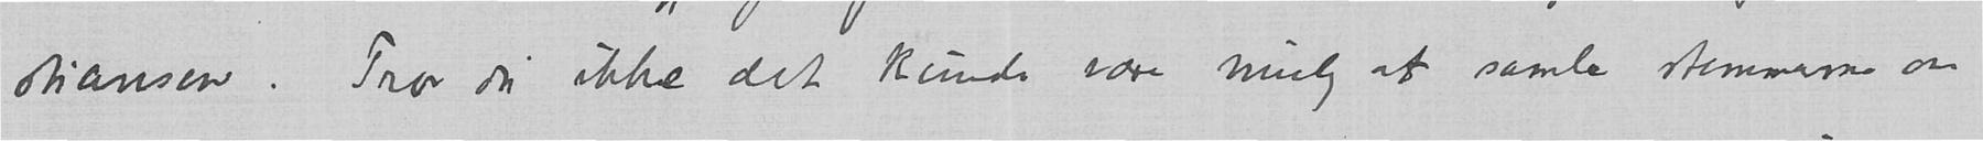tiansen. Tror du ikke det kunde være mulig at samle stemmerne om
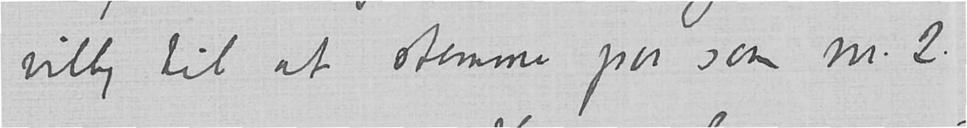villig til at stemme paa som nr. 2.
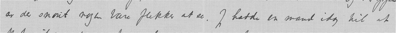er dert snaut nogen bare flekker at se. Jeg hadde en mand idag til at
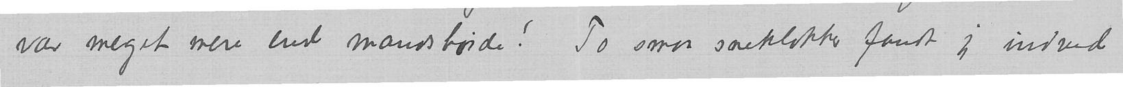var meget mere end mandshøide!	To smaa sneklokker fandt jeg indvend
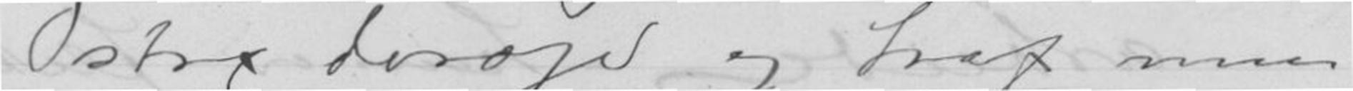strax derop og traf min
